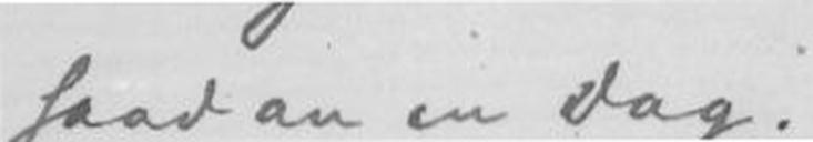saadan en Dag.
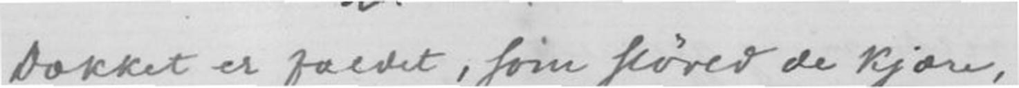Dækket er faldet, som sløred de Kjære,
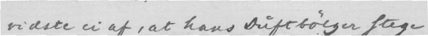vidste ei af, at hans Duftbølger stege
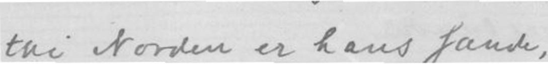thi Norden er hans sande,
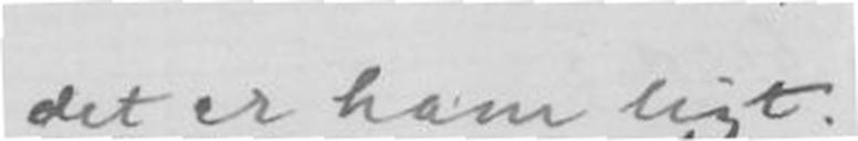det er ham ligt.
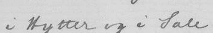i Hytter og i Sale
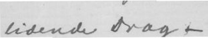lidende Drag -
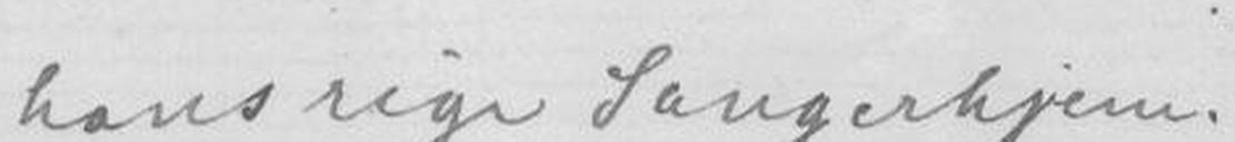hans rige Sangerhjem.
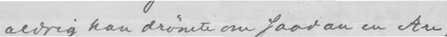aldrig han drømte om saadan en Ære,
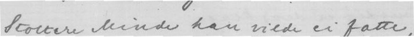Stoltere Minde han vilde ei fatte,
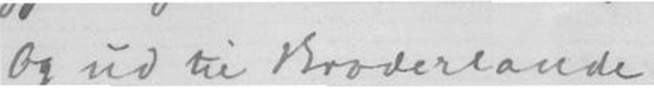Og ud til Broderlande
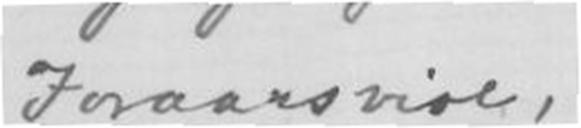Foraarsviole,
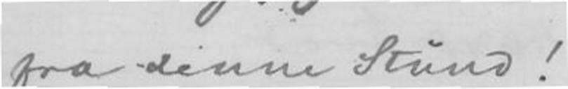fra denne Stund!
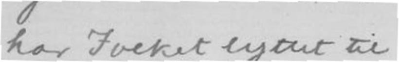har Folket lyttet til
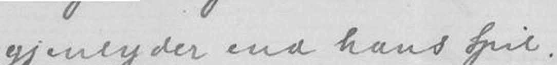gjenlyder end hans Spil.
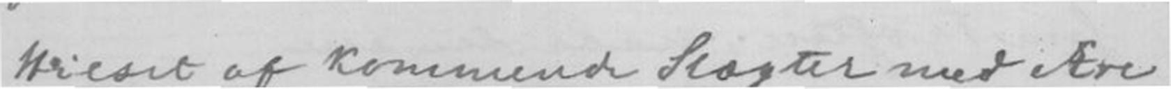Hilset af Kommende Slægter med Ære
